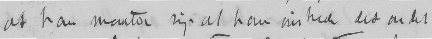at han maatte sige at han ønskede det andet
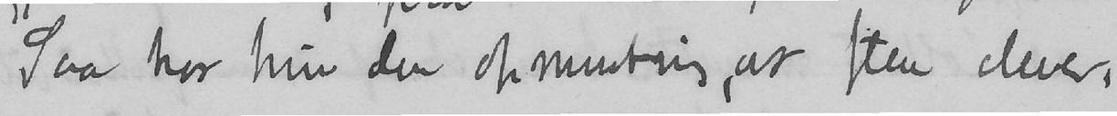Saa har hun den opmuntring, at flere elever,
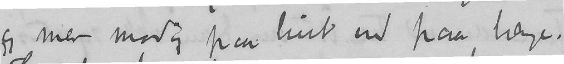og mer modig paa livet end paa længe.
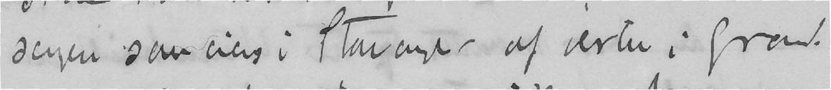sengen som eies i Stavanger af verten i Grand.
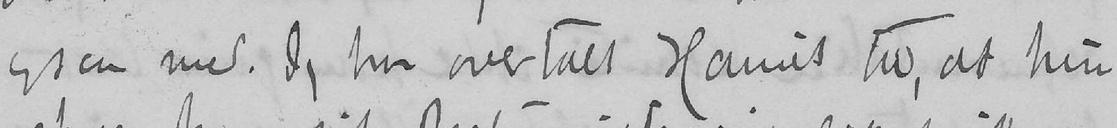ogsaa med. Jeg har overtalt Harriet til, at hun
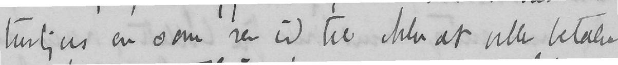turligvis en som ser ud til ikke at ville betale
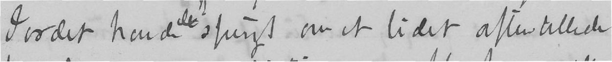Jordet havde de spurgt om et lidet aftenbillede
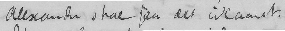Alexander skal faa det udlaanet.
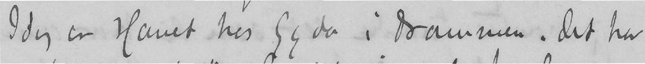Idag er Harriet hos Gyda i Drammen. Det har
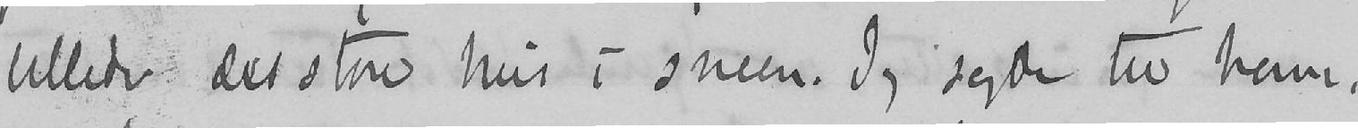billede det store hus i sneen. Jeg sagde til ham,
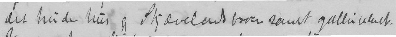det hvide hus og Skjævelandsbroen samt galleri billedet.
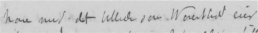have med det billede som Werenskiold eier
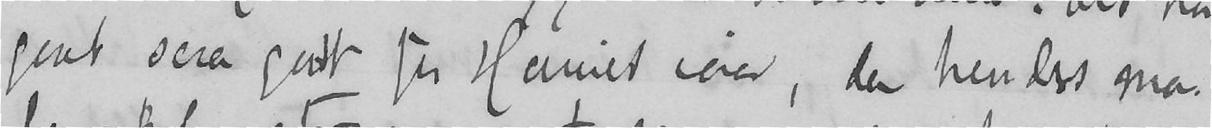gaat saa godt for Harriet iaar, da hendes ma-
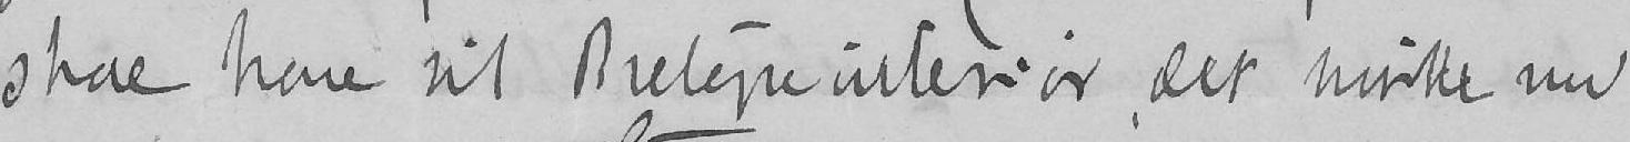skal have sit Bretagneinteriør, det mørke med
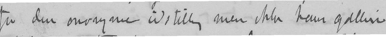fra den anonyme udstilling men ikke hans galleri
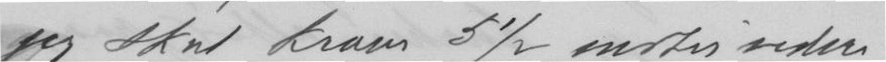jeg skal kræve 5 1/2 indtil videre,
